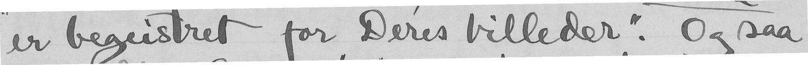er begeistret for Deres billeder". Og saa
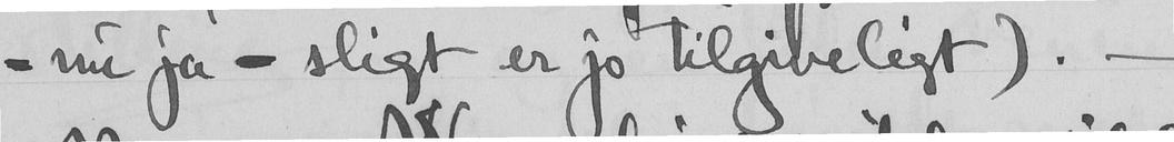- nu ja - sligt er jo tilgiveligt). -
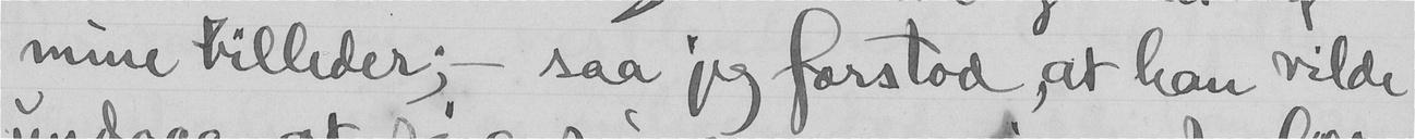mine billeder; - saa jeg forstod, at han vilde
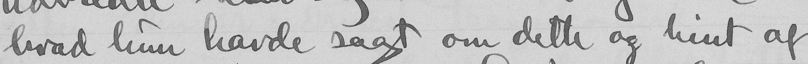hvad hun havde sagt om dette og hint af
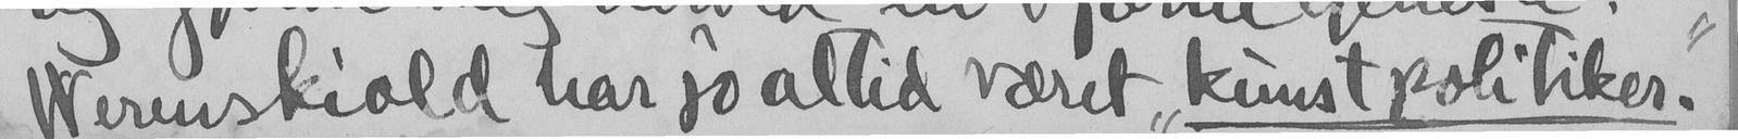Werenskiold har jo altid været "kunstpolitiker."
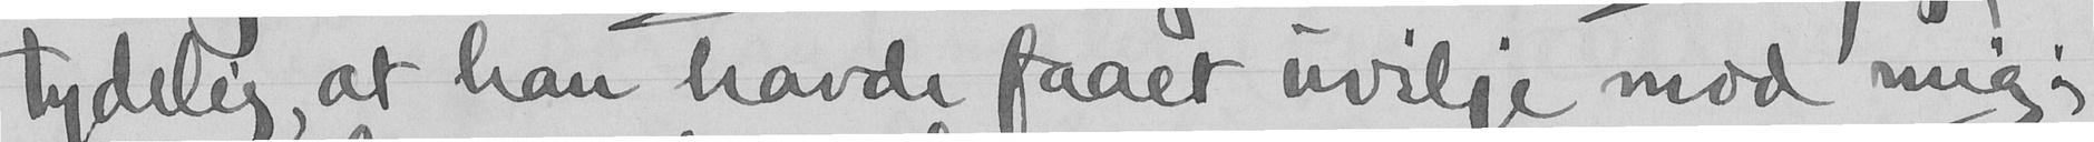tydelig, at han havde faaet uvilje mod mig;
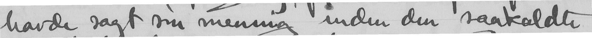havde sagt sin mening inden den saakaldte
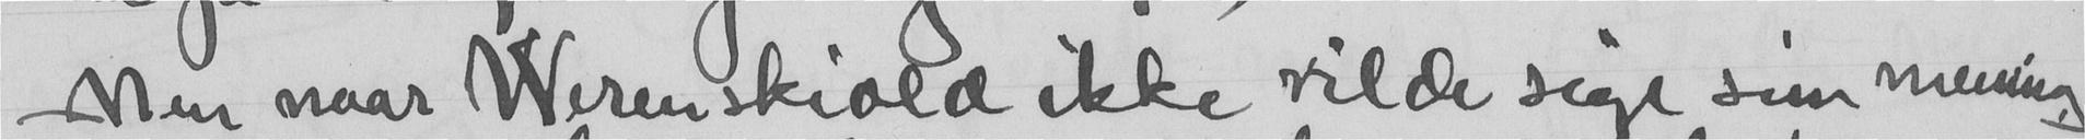Men naar Werenskiold ikke vilde sige sin mening
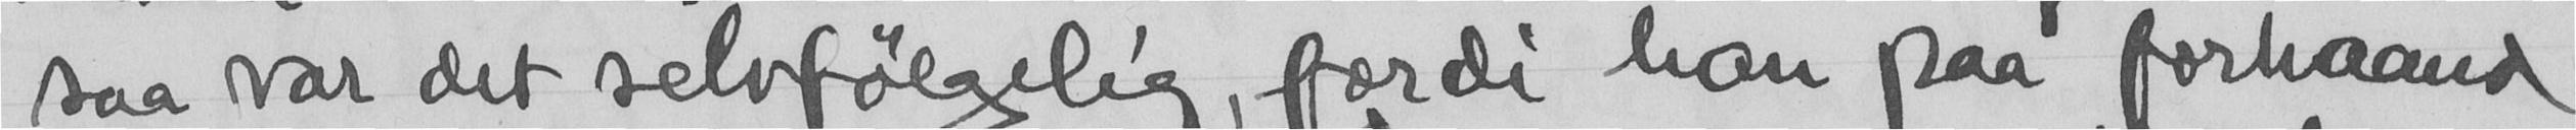saa var det selvfølgelig, fordi han paa forhaand
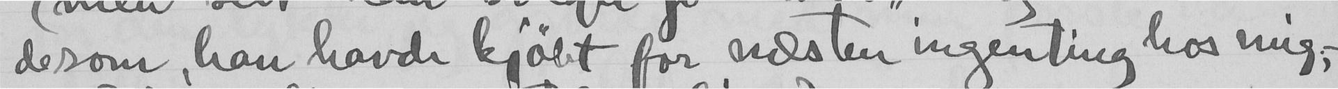de som, han havde kjøbt for næsten ingenting hos mig;
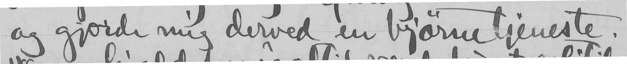og gjorde mig derved en bjørnetjeneste.
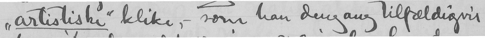"artistiske" klike, - som han dengang tilfældigvis
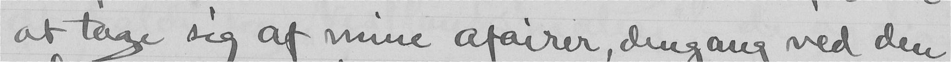at tage sig af mine afairer, dengang ved den
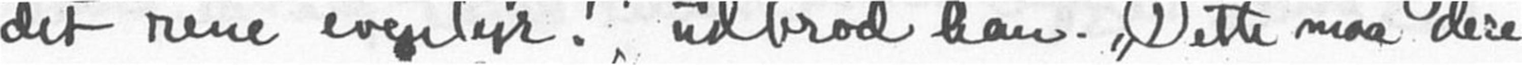det rene eventyr!" udbrød han. "Dette maa dere
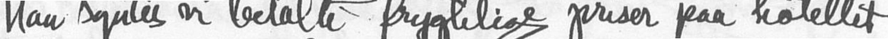Han syntes vi betalte frygtelige priser paa hotellet
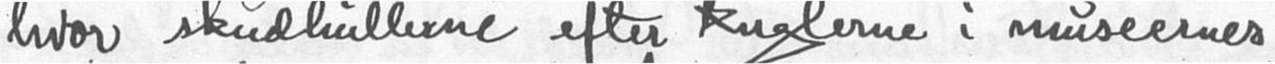hvor skudhullerne efter kuglerne i museernes
